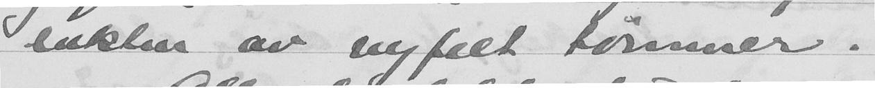lukten av nyfelt tømmer.
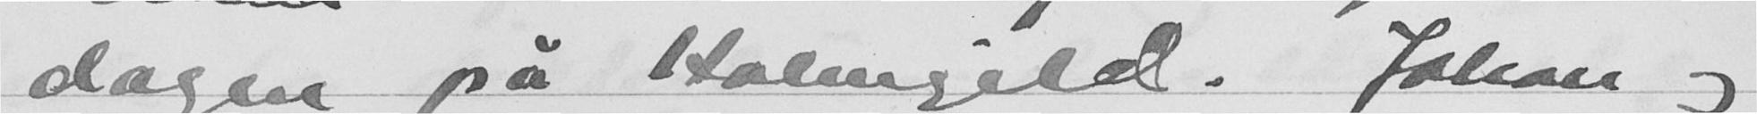dagen på Holmgild. Johan og
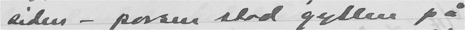siden - porsen stod gyllen på
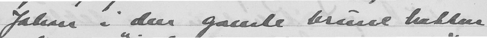Johan i den gamle brune hatten
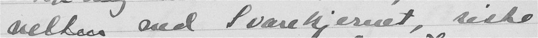velten ved Svankjernet, siste
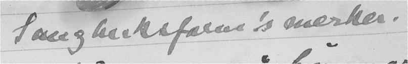Saugbusksforms merker.
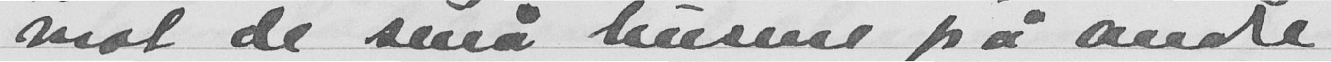mot de små husene på andre
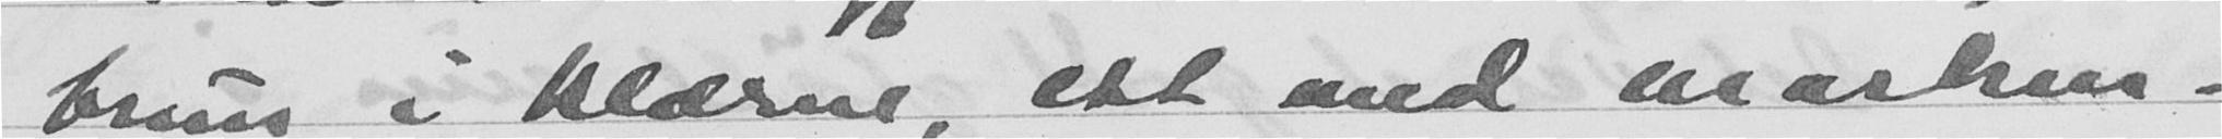brun i klærne, ett med masken.
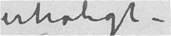utroligt –
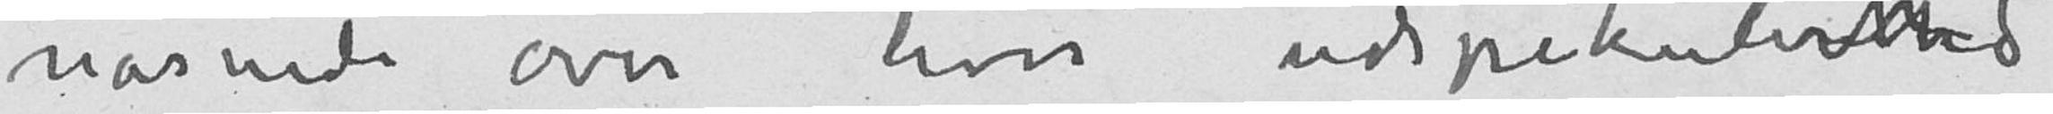rasende over hvor udspekulered
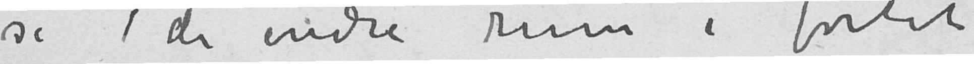sede indre rum i fortet
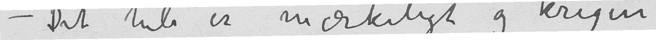– Det hele er mærkeligt og krigen
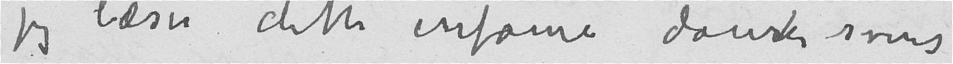jeg læser dette infame danske svins
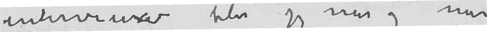interviewer blir jeg mer og mer
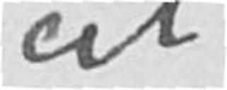at
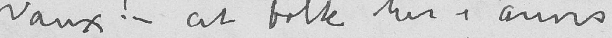Vaux! – at folk her i årevis
